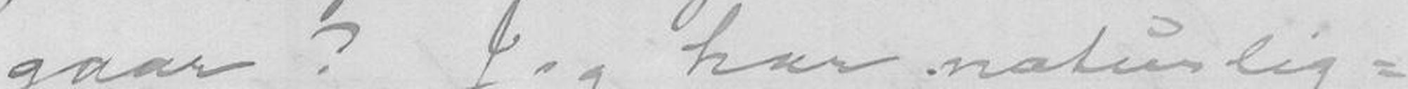gaar? Jeg har naturlig-
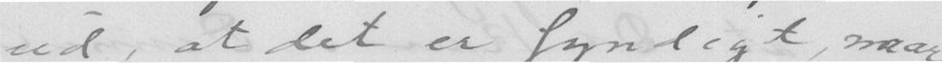ud, at det er syndigt, naar
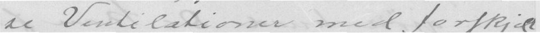se Ventilationer med forskjæ-
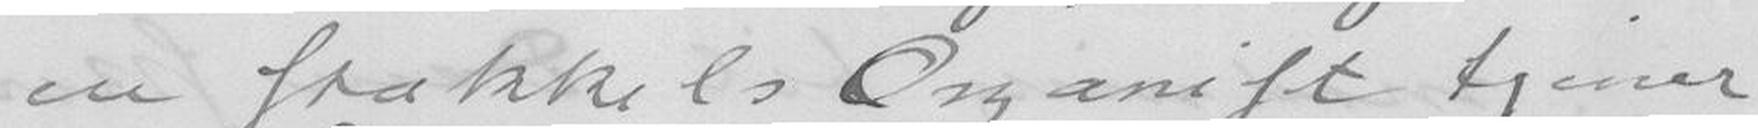en stakkels Organist tjener
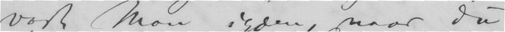vort Mon igjen, naar du
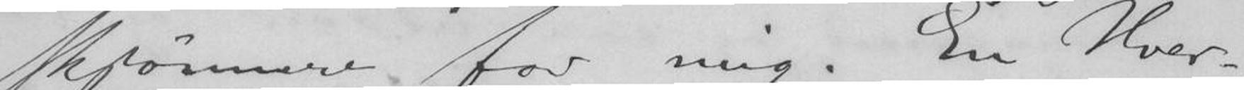skjønnere for mig. En Hver-
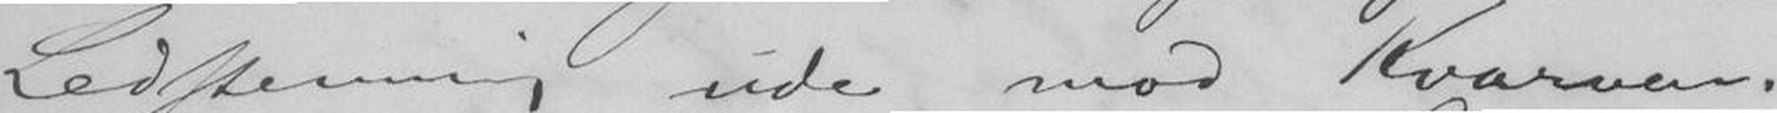Ledstemning ude mod Kvarven.
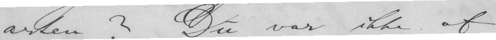arten? Du var ikke af
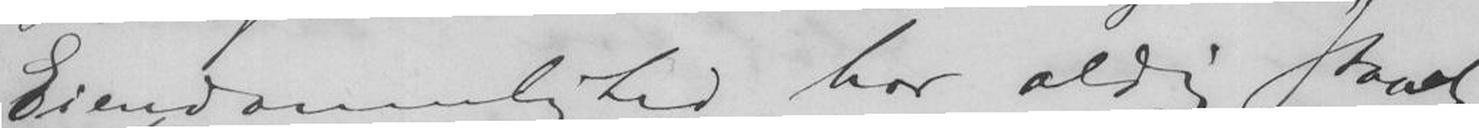Eiendommelighed har aldrig staaet
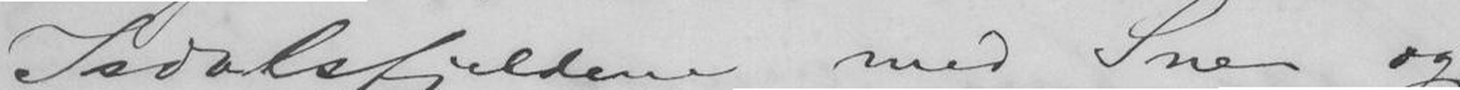Isdalsfjeldene med Sne og
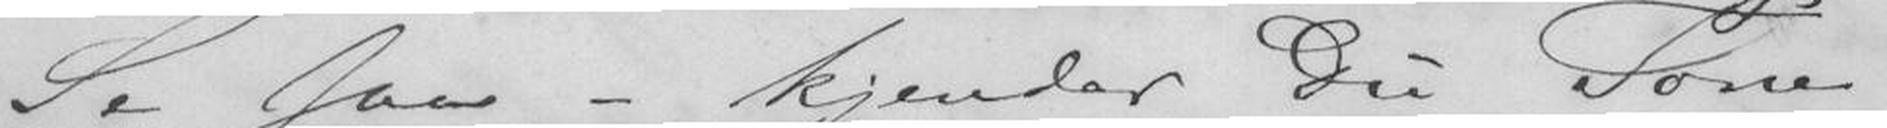Se saa - kjender Du Tone-
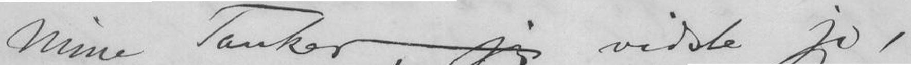mine Tanker, jeg vidste jo,
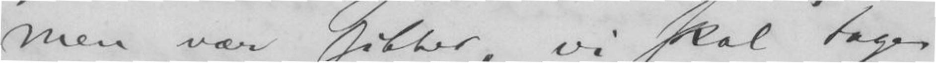men vær sikker, vi skal tage
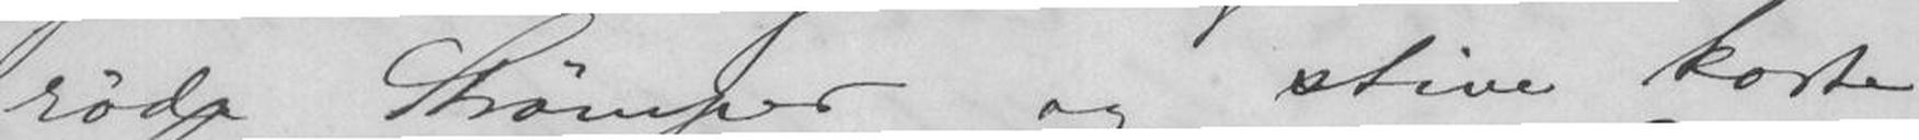røde Strømper og stive korte
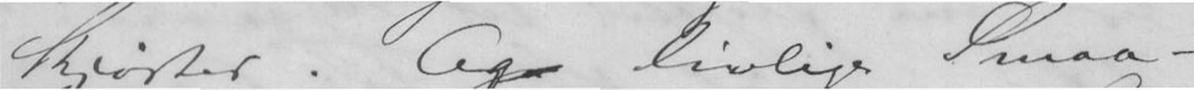Skjørter. Og livlige Smaa-
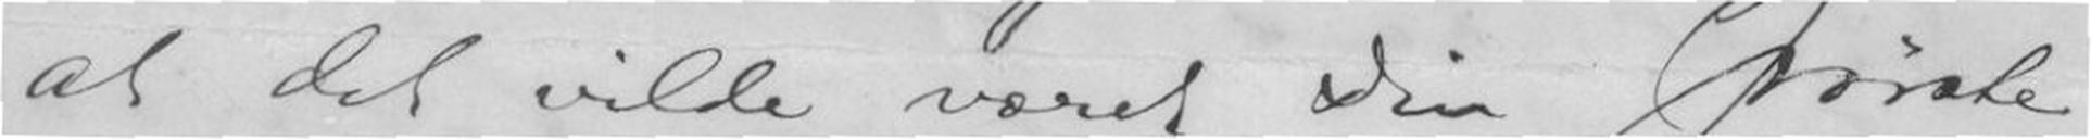at det ville været din største
